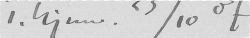T.hjem. 23/10 07
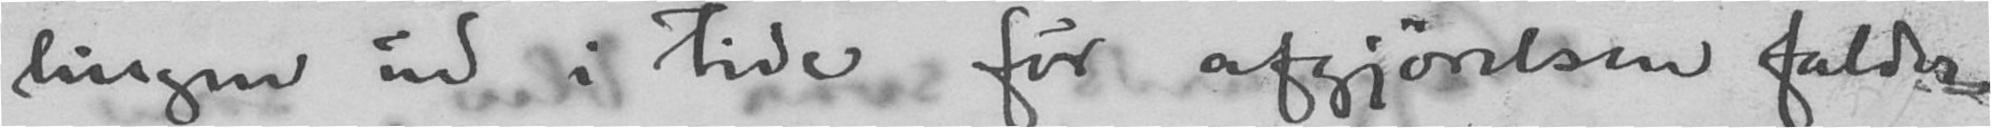lingen ud i tide før afgjørelsen falder.
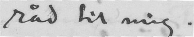råd til mig.
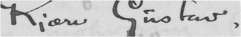Kjære Gustav,
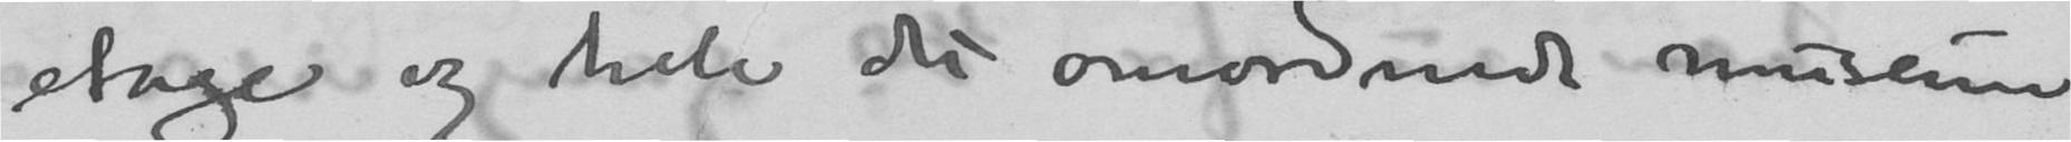etage og hele det omordnede museum
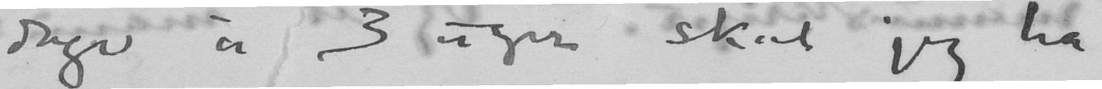dager à 3 uger skal jeg ha
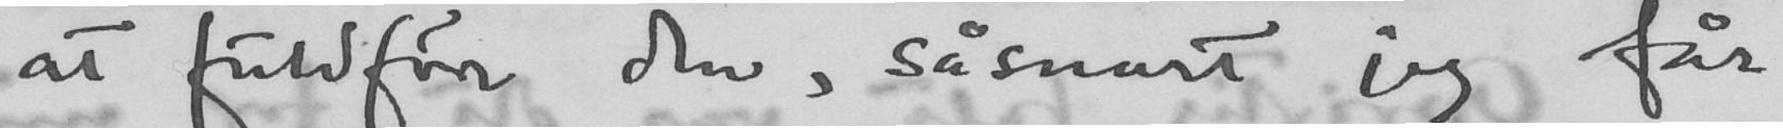at fuldføre den, så snart jeg får
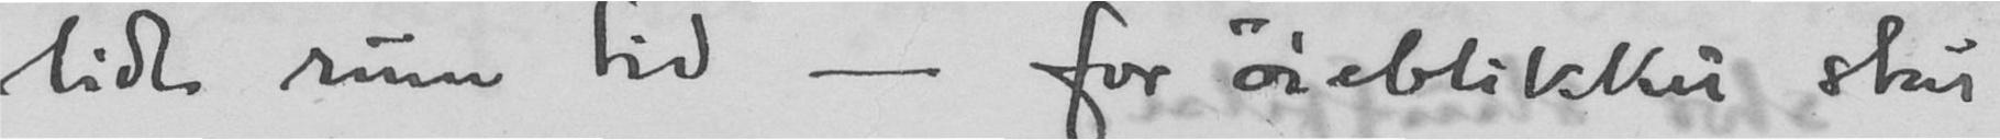lidt rum tid - for øieblikket står
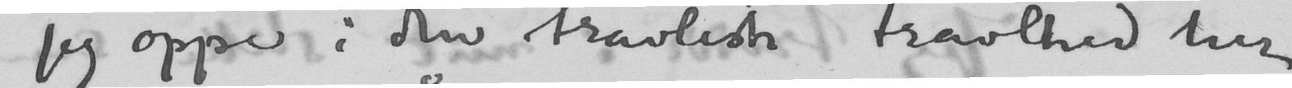jeg oppe i den travleste travlhed her
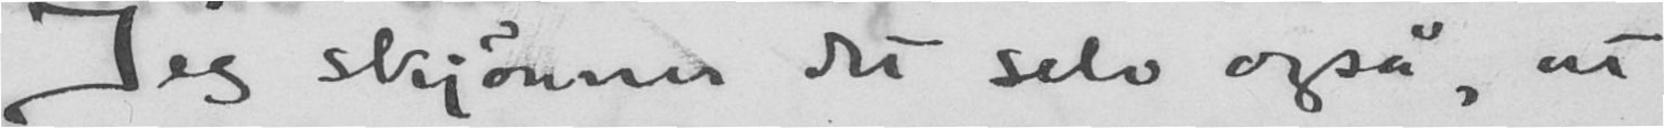Jeg skjønner det selv også, at
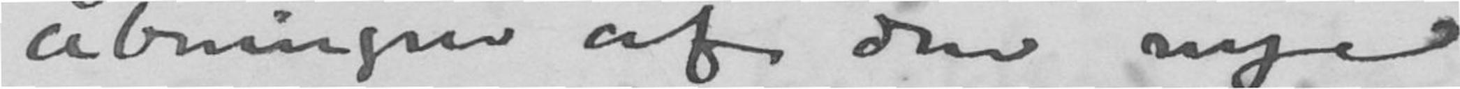åbningen af den nye
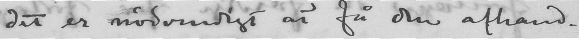det er nødvendigt at få den afhand-
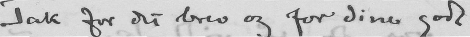Tak for det brev og for dine gode
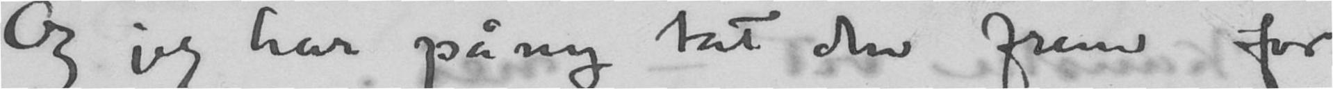Og jeg har på ny tat den frem for
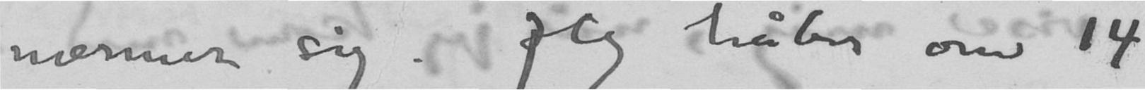nærmer sig. Jeg håber om 14
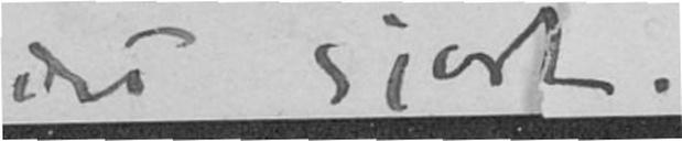det gjort.
